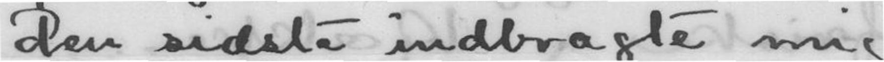Den sidste indbragte mig
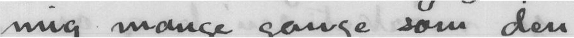mig mange gange som den
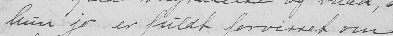hun jo er fuldt forvisset om
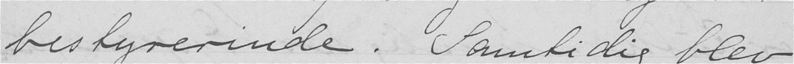bestyrerinde. Samtidig blev
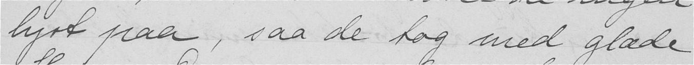lyst paa, saa de tog med glæde
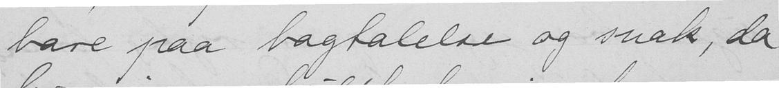bare paa bagtalelse og snak, da
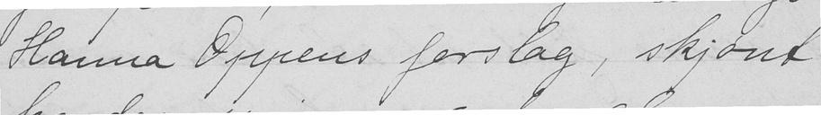Hanna Oppens forslag, skjønt
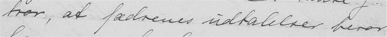tror, at fædrenes udtalelser beror
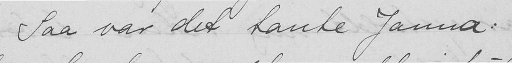Saa var det tante Janna.
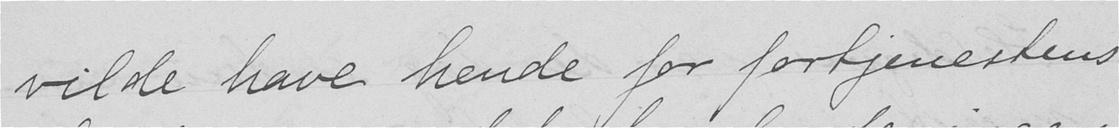vilde have hende for fortjenestens
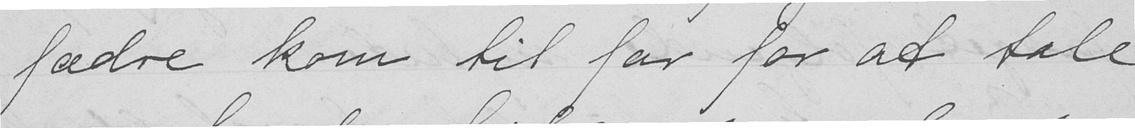fædre kom til far for at tale
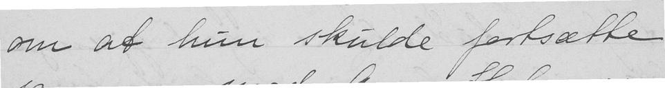om at hun skulde fortsætte
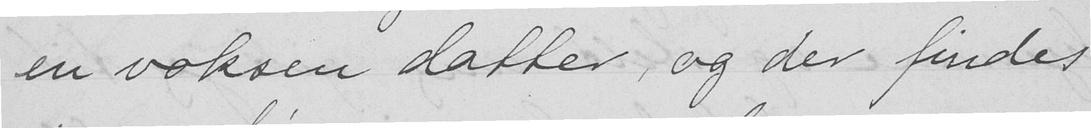en voksen datter, og der findes
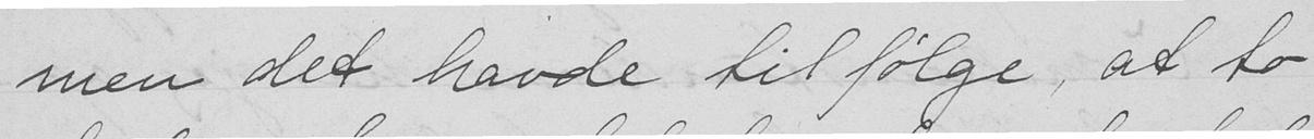men det havde til følge, at to
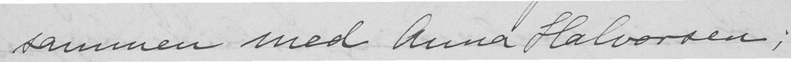sammen med Anna Halvorsen;
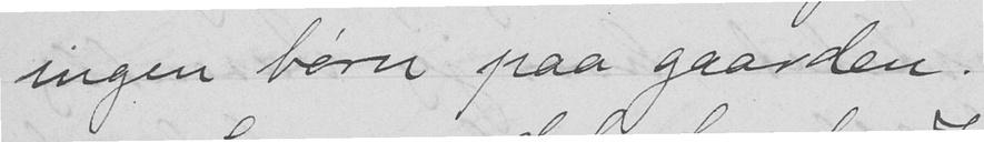ingen børn paa gaarden.
